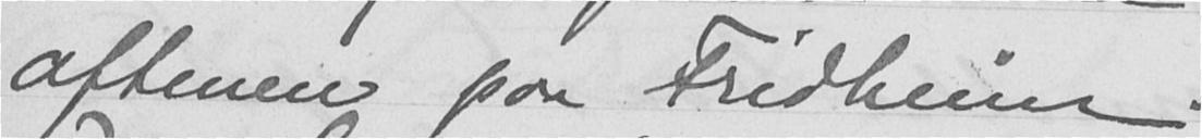aftenen paa Fridheim.
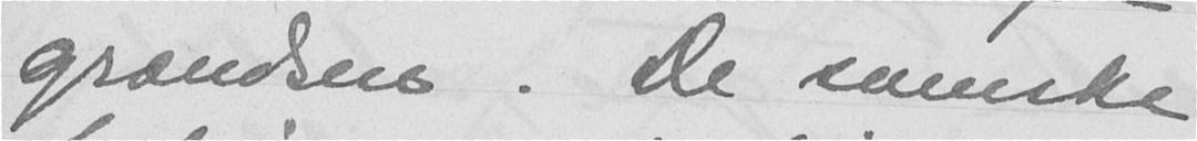grændsen. De svenske
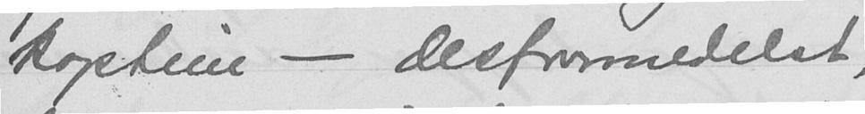kaptein - desformedelst,
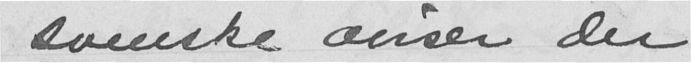svenske aviser der
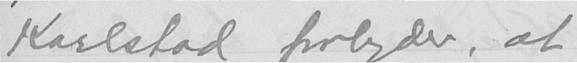Karlstad forlyder, at
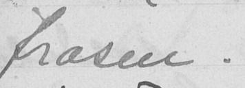frasen.
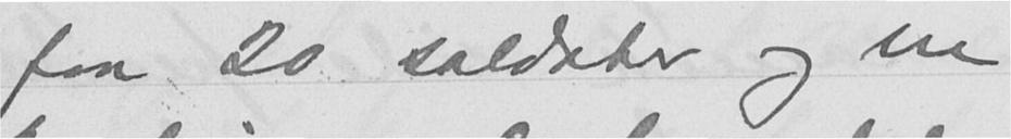faa 20 soldater og en
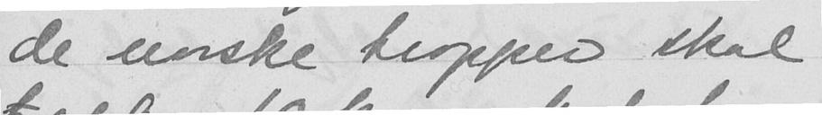de norske tropper skal
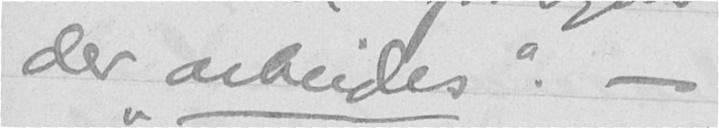der "arbeides". -
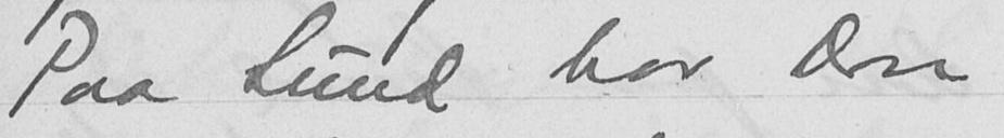Paa Sund har Don
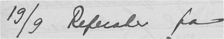19/9 Referater fra
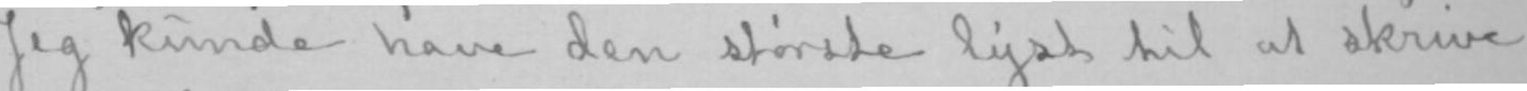Jeg kunde have den største lyst til at skrive
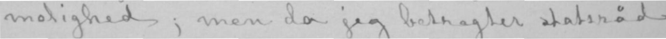melighed; men da jeg betragter statsråd
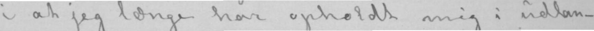i at jeg længe har opholdt mig i udlan-
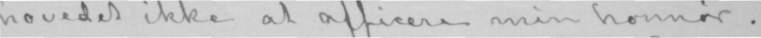hovedet ikke at afficere min honnør.
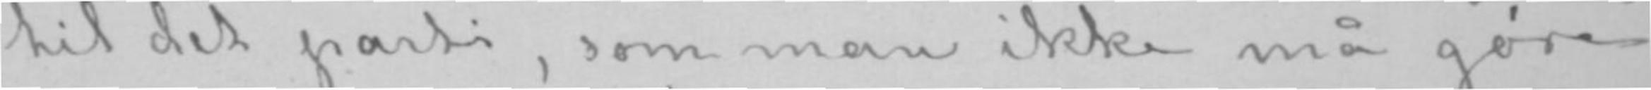til det parti, som man ikke må gøre
